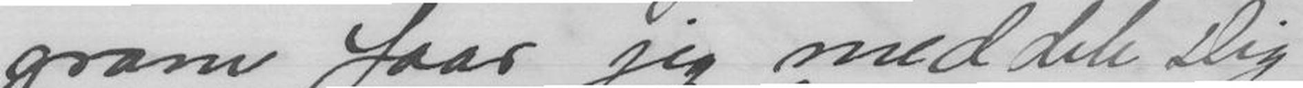gram faar jeg meddele Dig
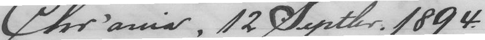Chr'ania, 12 Septbr. 1894.
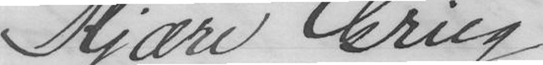Kjære Grieg
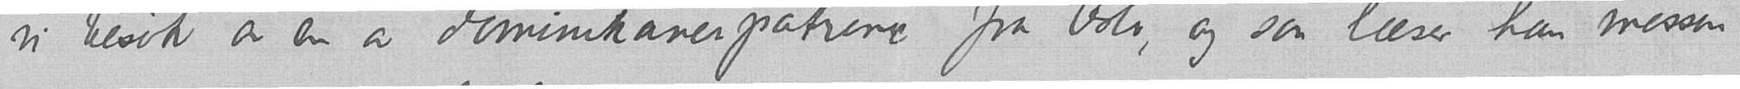vi besøk av en av dominikanerpatrene fra Oslo, og saa læser han messen
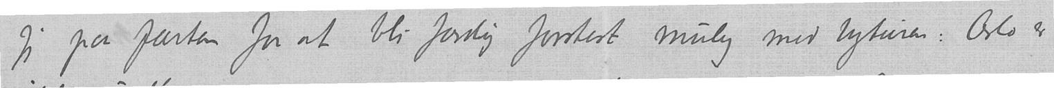jeg paa farten for at bli færdig fortest mulig med byturen: Oslo er
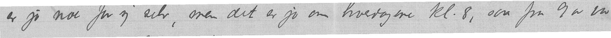er jo noe for sig selv, men det er jo om hverdagene kl. 8, saa fra 9 av var
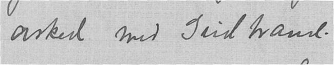avsked med Gudbrand.
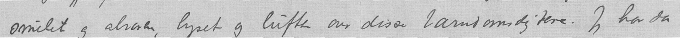smilet og alvoren, lyset og luften over disse barndomsdigtene. Jeg har da
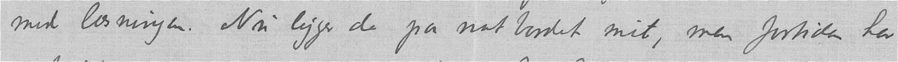med læsningen. Nu ligger de paa natbordet mit, men fortiden har
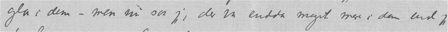gla i dem – men nu saa jeg, der var endda meget mere i dem end jeg
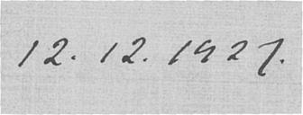12.12.1927.
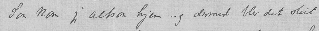Saa kom jeg altsaa hjem – og dermed blev det slut
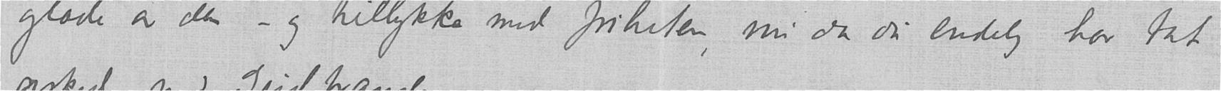glæde av den – og tillykke med friheten, nu da du endelig har tat
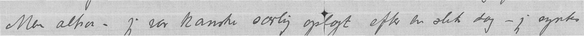Men altsaa – jeg var kanske særlig oplagt efter en slik dag – jeg syntes
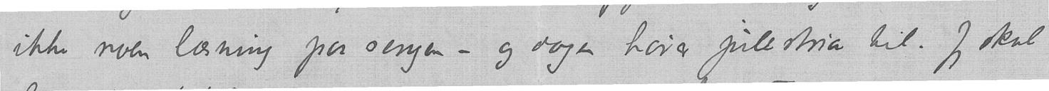ikke noen læsning paa sengen – og dagen hører julestria til. Jeg skal
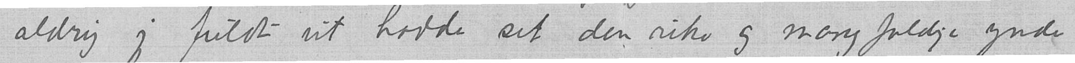aldrig jeg fuldt ut hadde set den rike og mangfoldige ynde,
